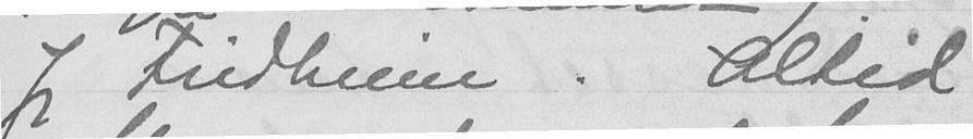Jeg Fridheim. Altid
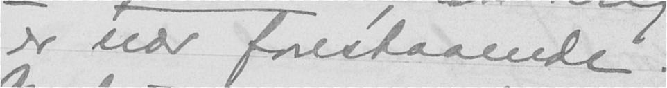er nær forestaande.
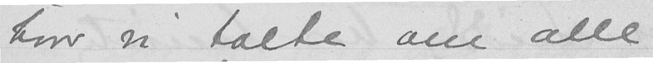hvor vi talte om alle
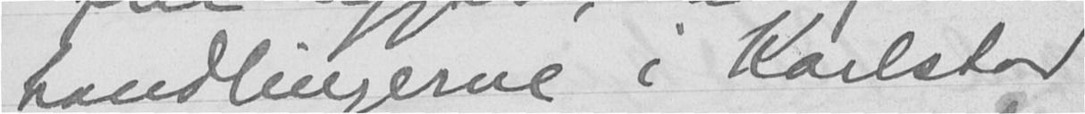handlingerne i Karlstad
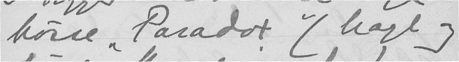bøsse "Paradot" (hagl og
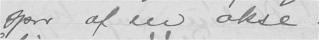spor af en okse.
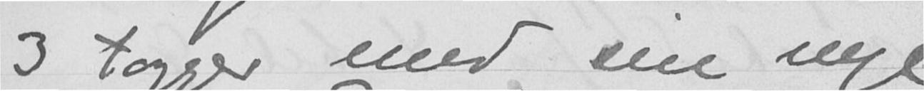3 tagger med sin nye
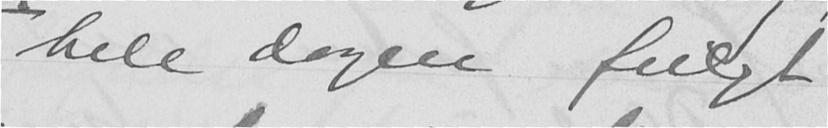hele dagen fulgt
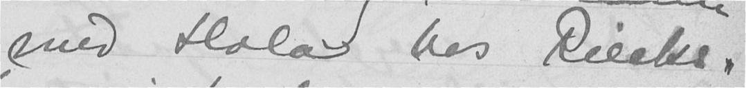med Hola hos Riecks,
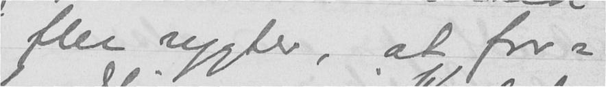fler rygter, at for-
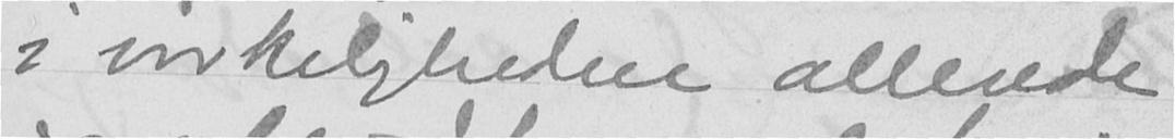i virkeligheden allerede
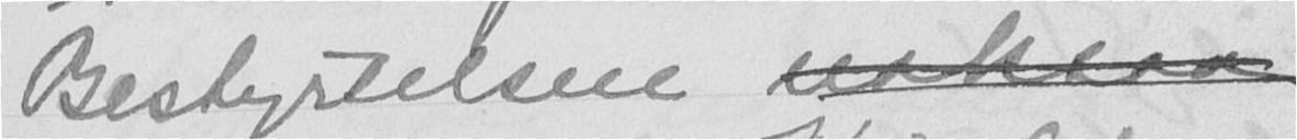Bestyrelsen noksaa
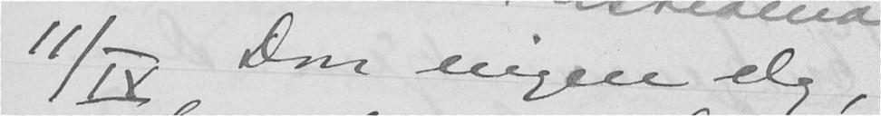11/IX Don ingen elg,
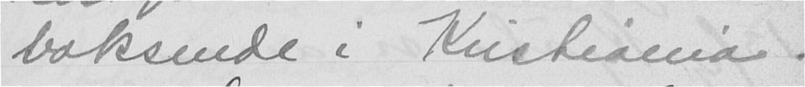baksende i Kristiania.
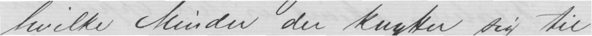hvilke Minder der knytter sig til
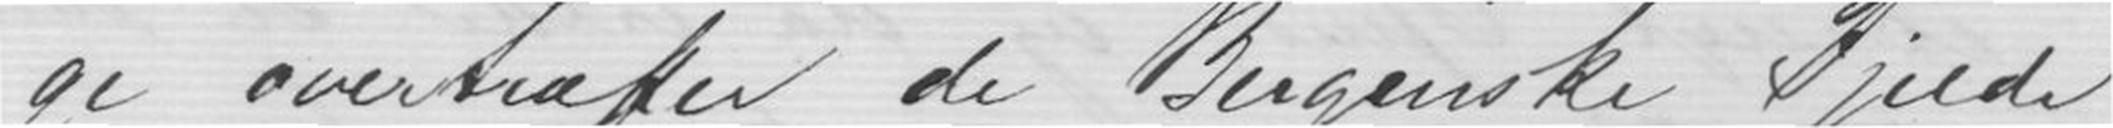ge overtræffer de Bergenske Fjelde
